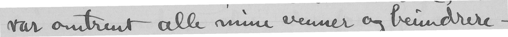var omtrent alle mine venner og beundrere -
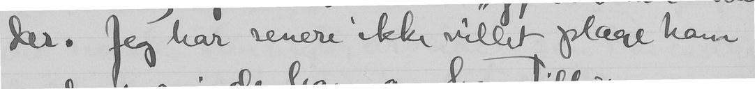der. Jeg har senere ikke villet plage ham
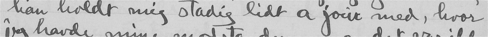han holdt mig stadig lidt a jour med, hvor
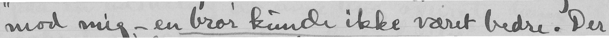mod mig, - en bror kunde ikke været bedre. Der
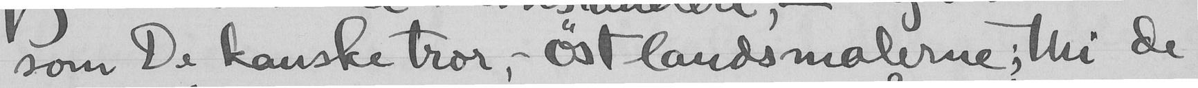som De kanske tror, - østlandsmalerne; thi de
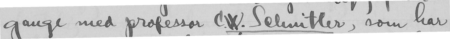gange med professor C.W. Selmitler, som har
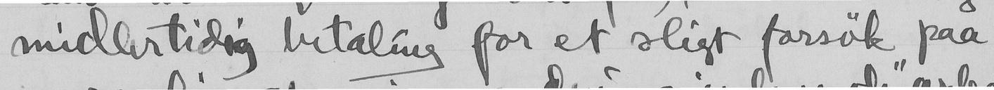midlertidig betaling for et sligt forsøk paa
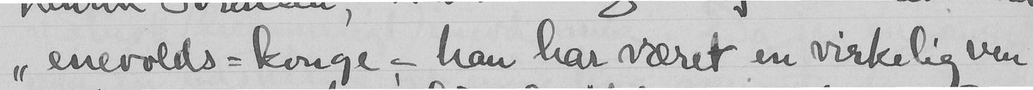"enevolds-konge", - han har været en virkelig ven
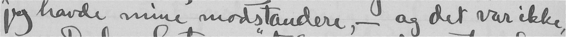jeg havde mine modstandere, - og det var ikke,
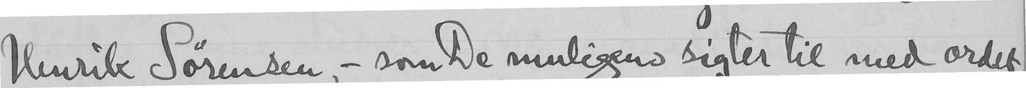Henrik Sørensen - som De muligens sigter til med ordet
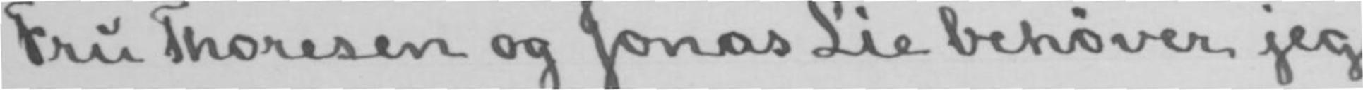Fru Thoresen og Jonas Lie behøver jeg
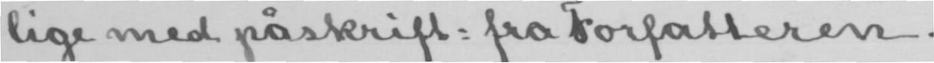lige med påskrift: fra Forfatteren.
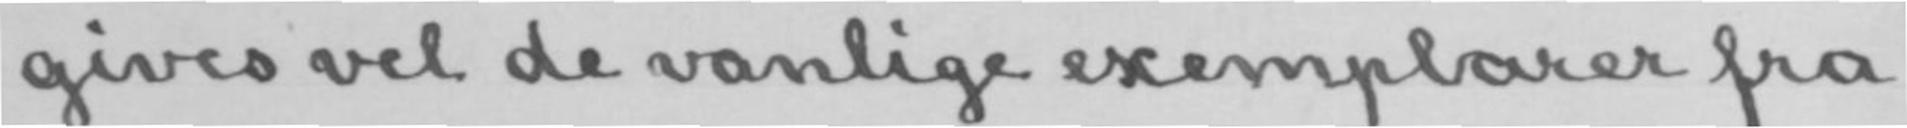gives vel de vanlige exemplarer fra
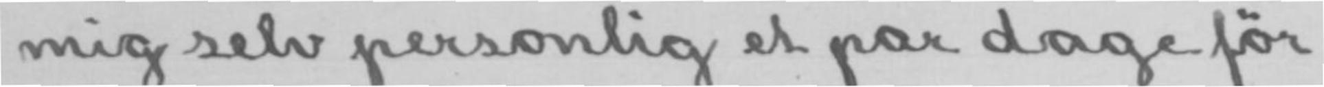mig selv personlig et par dage før
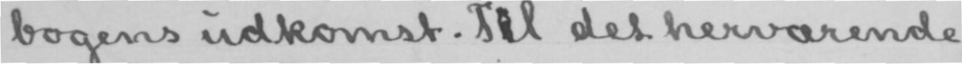bogens udkomst. Tlil det herværende
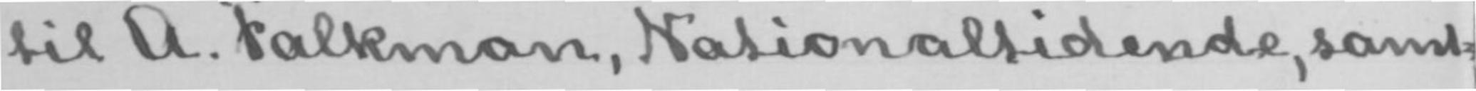til A. Falkman, Nationaltidende, samt-
